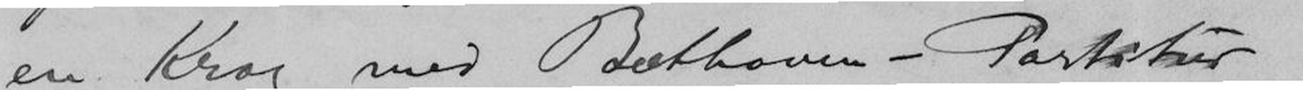en Krog med Beethoven-Partitur
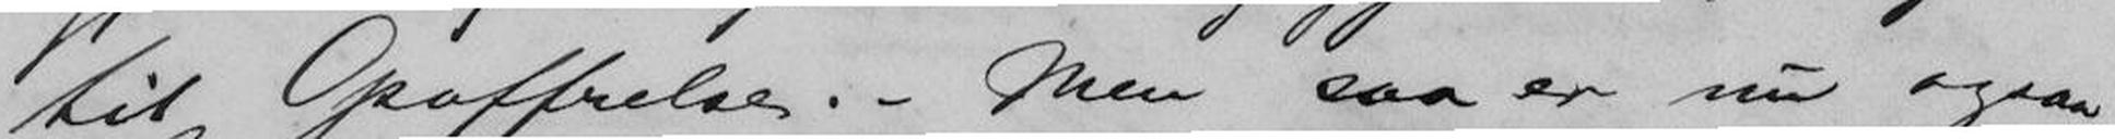til Opoffrelse. - Men saa er nu ogsaa
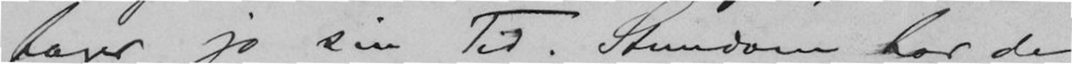tager jo sin Tid, Stundom har de
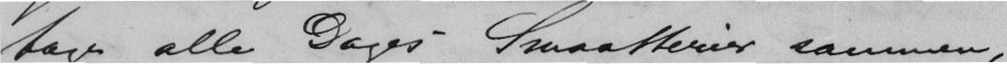tage alle Dages Smaatterier sammen,
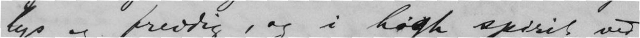lys og freidig, og i high spirit ved
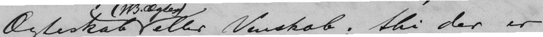Ægteskab eller Venskab; thi der er
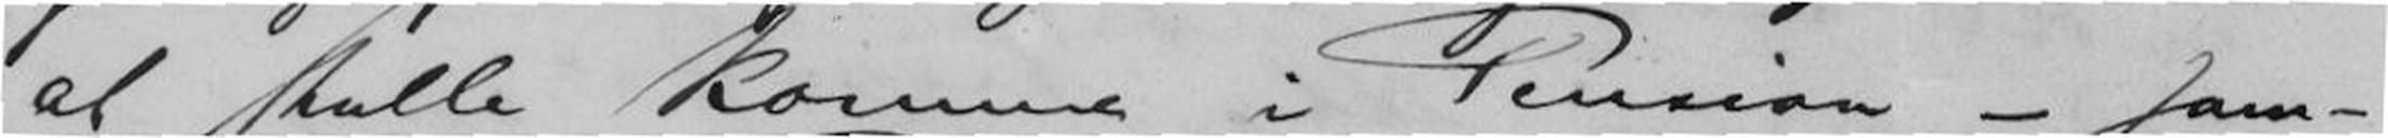at skulle komme i Pension - sam-
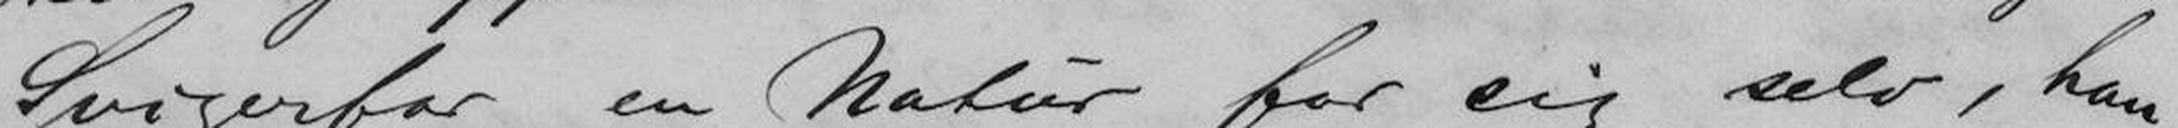Svigerfar en Natur for sig selv, han
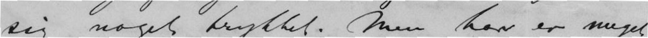sig noget trykket. Men han er meget
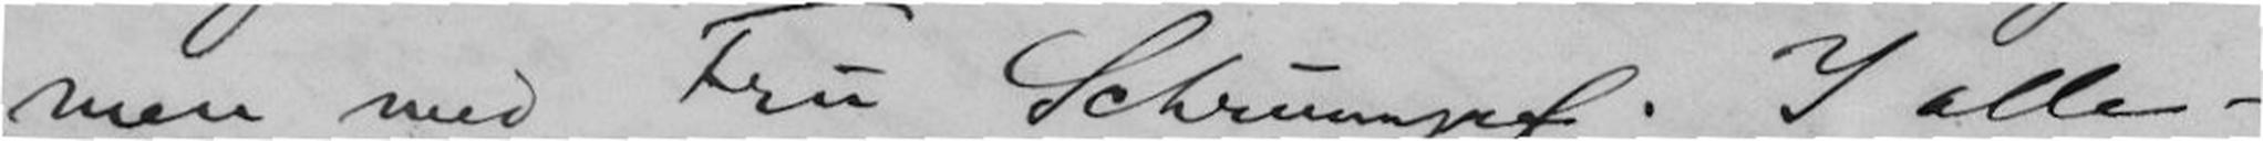men med Fru Schrumpf. I alle-
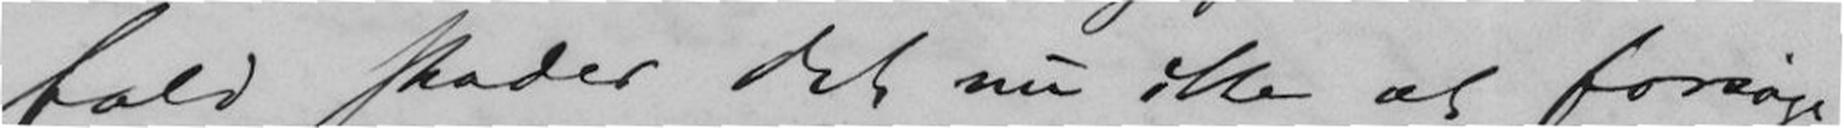fald skader det nu ikke at forsøge.
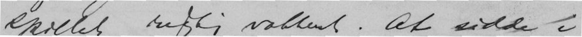spillet rigtig vakkert. At sidde i
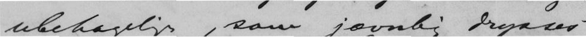ubehagelige, som jævnlig drysses
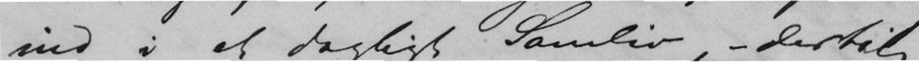ind i et dagligt Samliv, - dertil
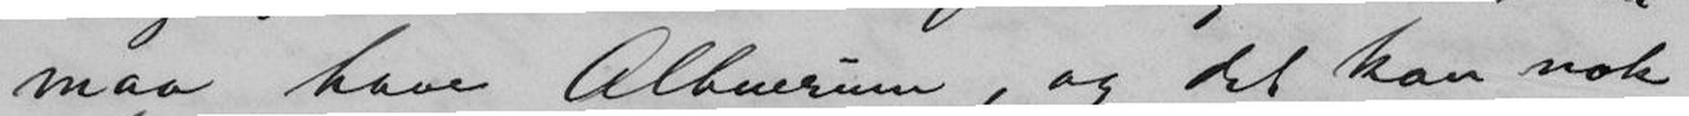maa have Albuerum, og det kan nok
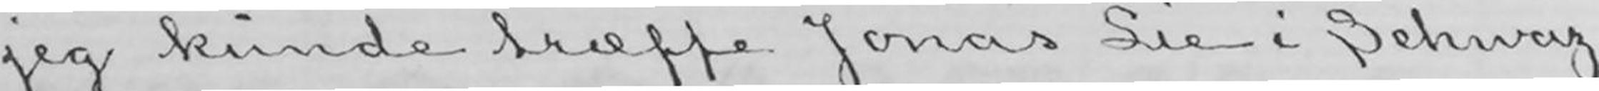jeg kunde træffe Jonas Lie i Schwaz;
